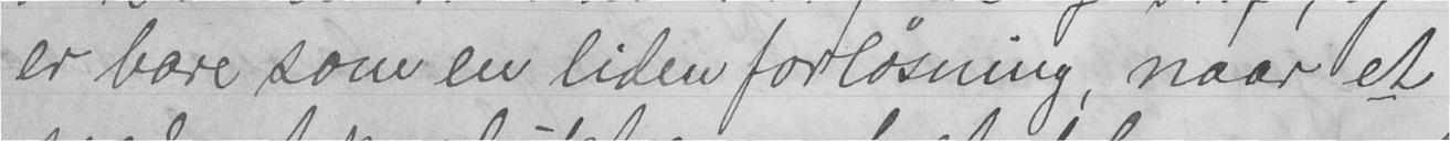er bare som en liden forløsning, naar et
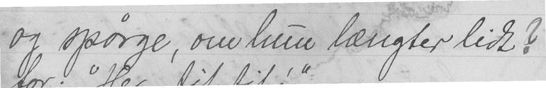og spørge, om hun længter lidt?
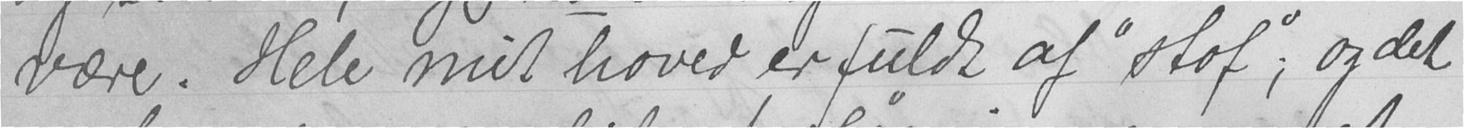være. Hele mit hoved er fuldt af "stof"; og det
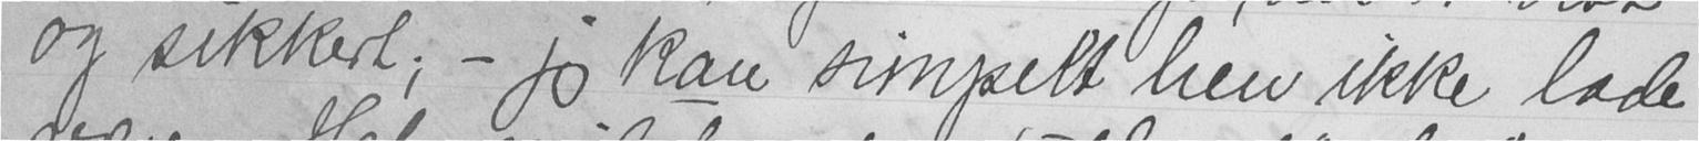og sikkert; - jeg kan simpelt hen ikke lade
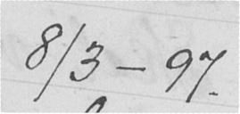8/3-97.
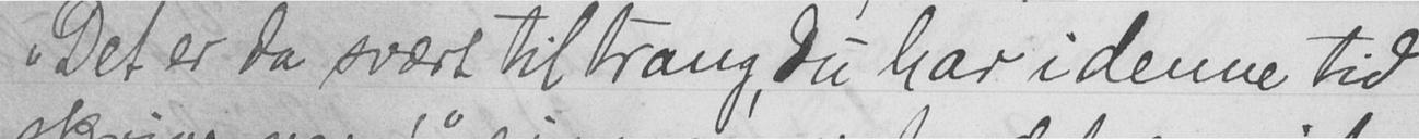"Det er da svært til trang, du har i denne tid
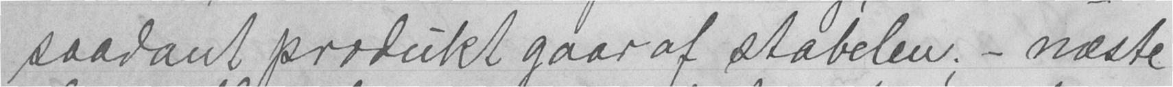saadant produkt gaar af stabelen; - næste
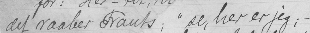det raaber Frants; "se, her er jeg; -
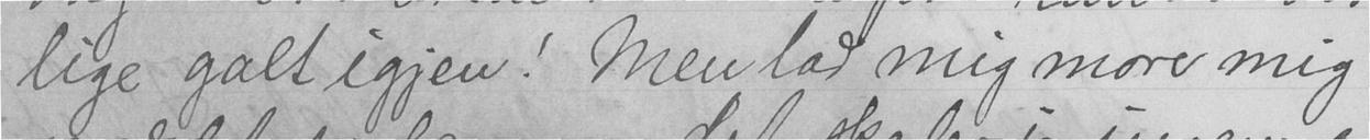lige galt igjen! Men lad mig more mig
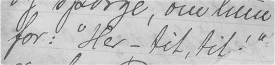for: "Her - tit, tit!"
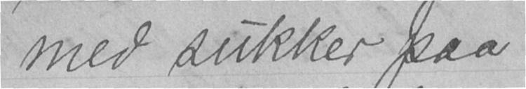med sukker paa
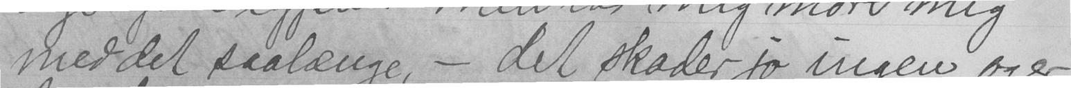med det saalænge, - det skader jo ingen og er
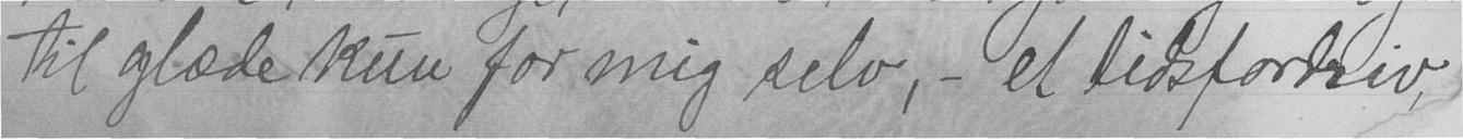til glæde kun for mig selv, - et tidsfordriv
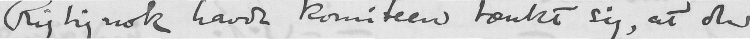Rigtignok havde komiteen tænkt sig, at de
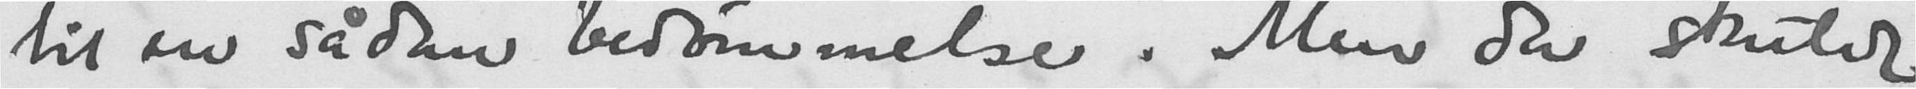til en sådan bedømmelse. Men da skulde
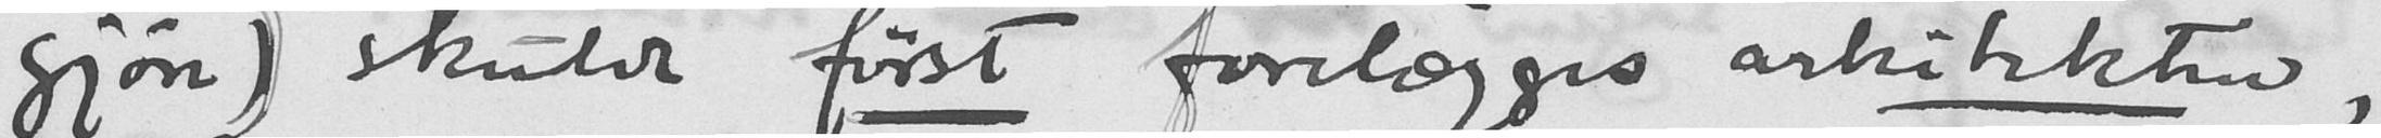gjøre) skulde først forelægges arkitekten,
